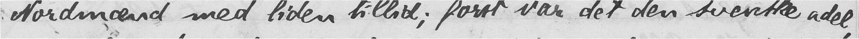Nordmænd med liden tillid; først var det den svenske adel,
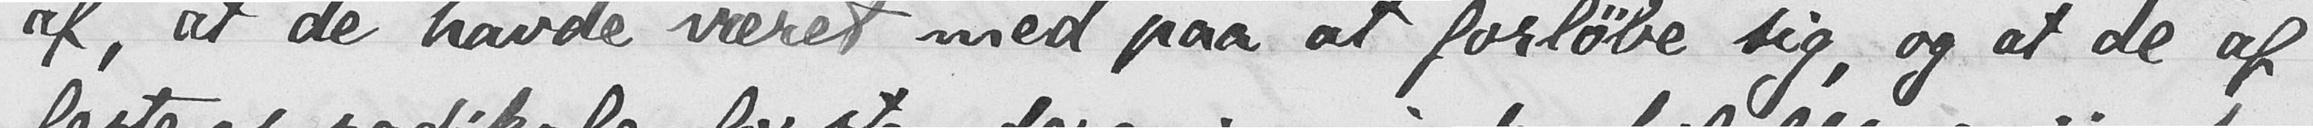af, at de havde været med paa at forløbe sig, og at de af
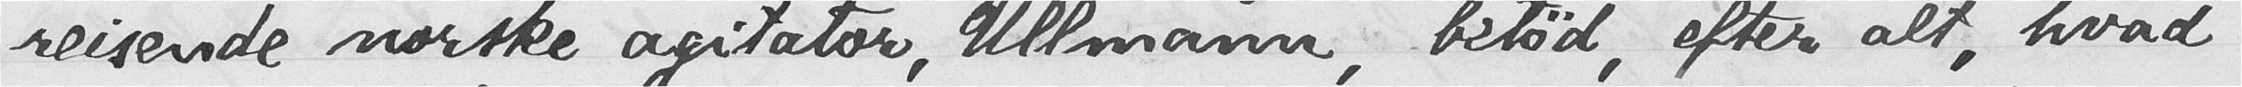reisende norske agitator, Ullmann, betød, efter alt, hvad
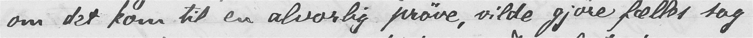om det kom til en alvorlig prøve, vilde gjøre fælles sag
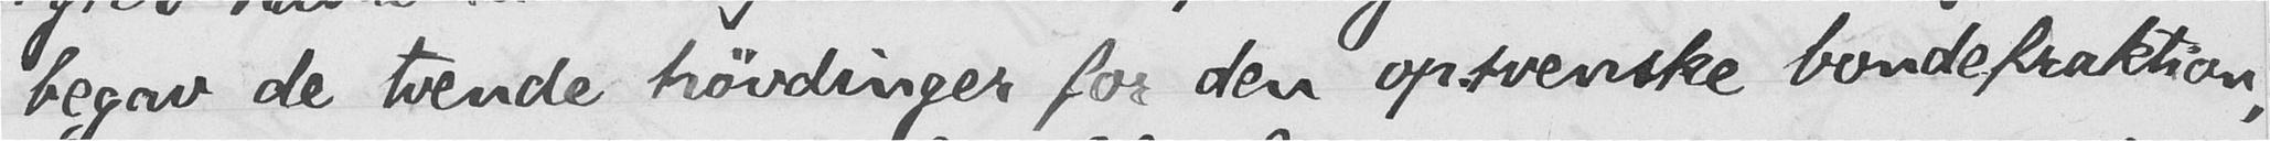begav de tvende høvdinger for den opsvenske bondefraktion,
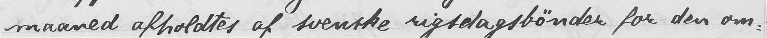maaned afholdtes af svenske rigsdagsbønder for den om-
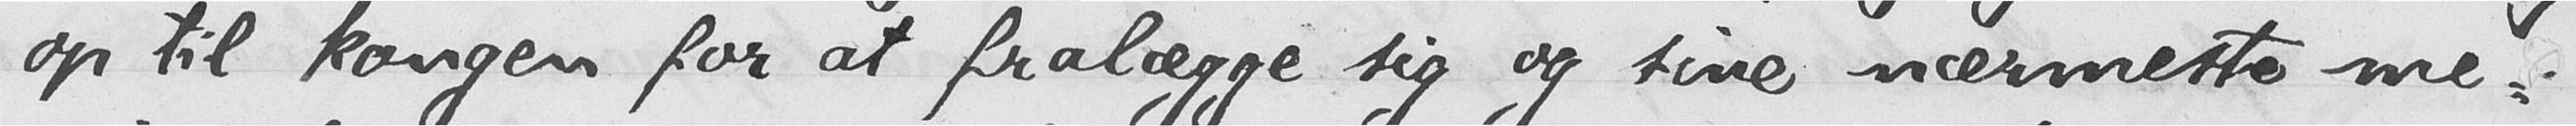op til kongen for at fralægge sig og sine nærmeste me-
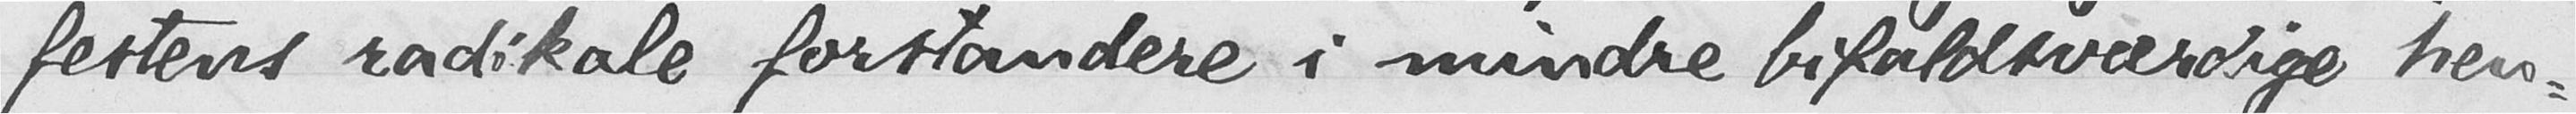festens radikale forstandere i mindre bifaldsværdige hen-
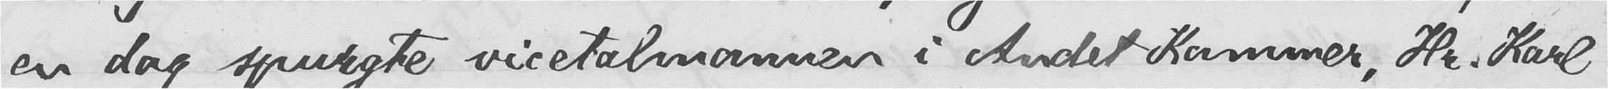en dag spurgte vicetalsmannen i Andet Kammer, Hr. Karl
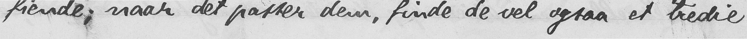fiende; naar det passer dem, finde de vel ogsaa et tredie
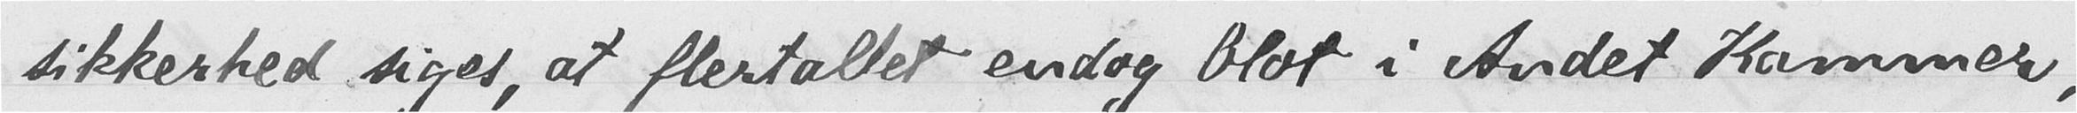sikkerhed siges, at flertallet endog blot i Andet Kammer,
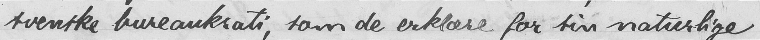svenske bureaukrati, som de erklære for sin naturlige
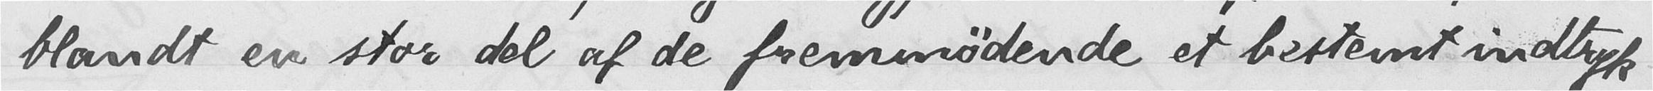blandt en stor del af de fremmødende et bestemt indtryk
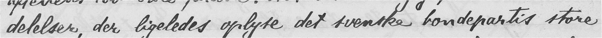delelser, der ligeledes oplyse det svenske bondepartis store
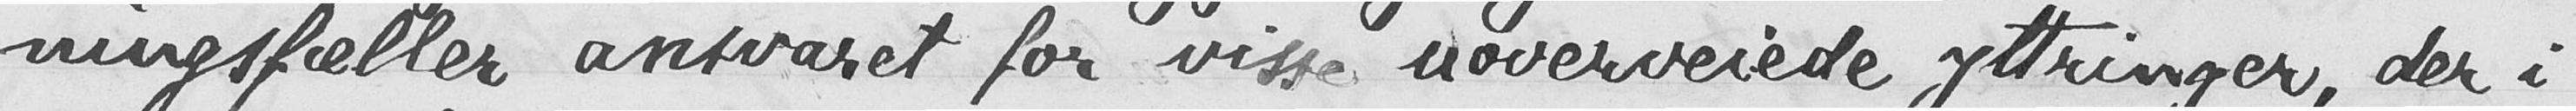ningsfæller ansvaret for visse uoverveiede yttringer, der i
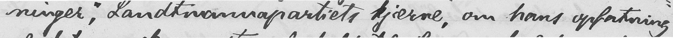ninger", Landtmannapartiets kjærne, om hans opfatning
